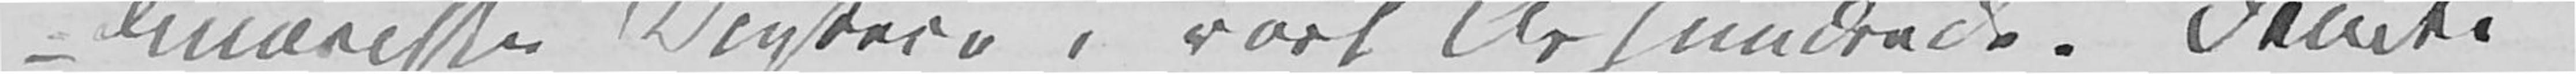dinaviske Digtere i vort Århundrede». Femti
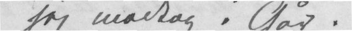jeg modtog i Går.
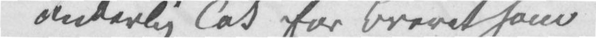Inderlig Tak for Brevet som
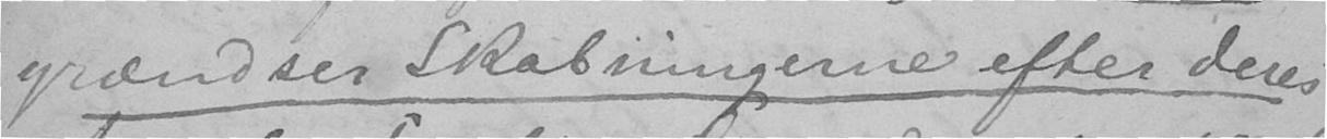grændser Skabningerne efter deres
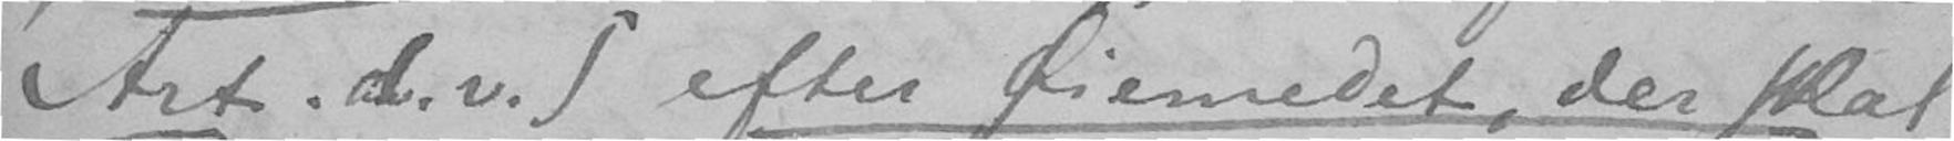Art. d.v.s. efter Øiemedet, der skal
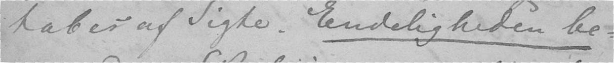tabes af Sigte. Endeligheden be-
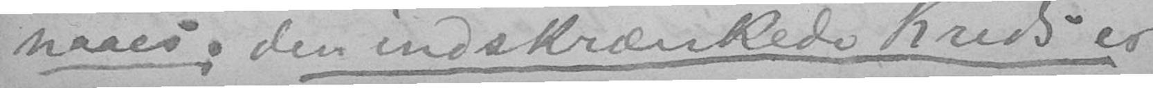naaes; den indskrænkede Kreds er
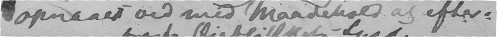opnaaes ved med Maadehold og efter-
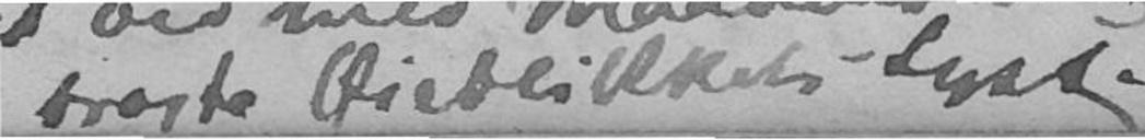tragte Øieblikket Lyst.
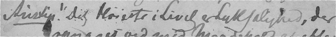Anlig. "Det høieste i Livet er Lyksalighed, der
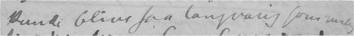kunde blive saa langvarig som mulig
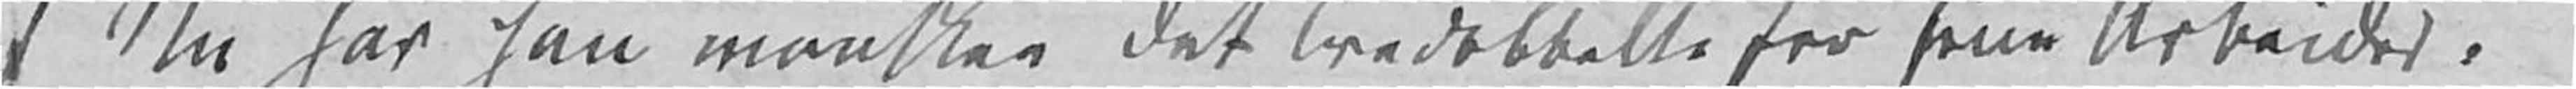Nu har han maaskee det Tredobbelte for sine Arbeider.
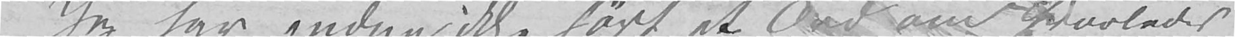Jeg har endnu ikke hørt et Ord om hvorledes
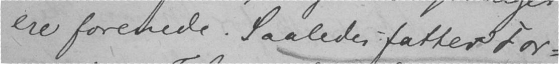ere forenede. Saaledes fatter For-
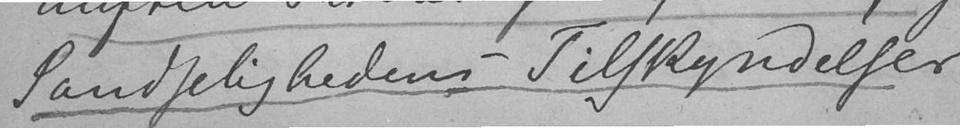Sandselighedens Tilskyndelser
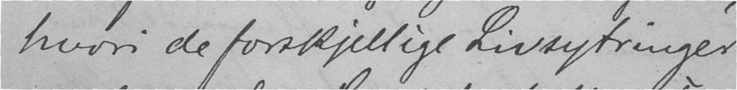hvori de forskjellige Livsytringer
